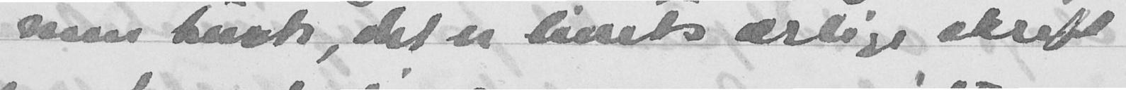men husk, det er livets ærlige skrift
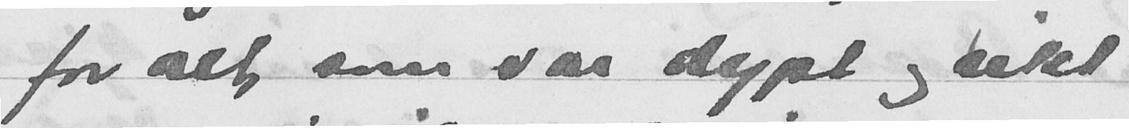for alt, som var dypt og rikt
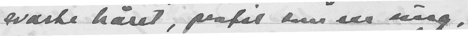svarte håret, profil som en ung,
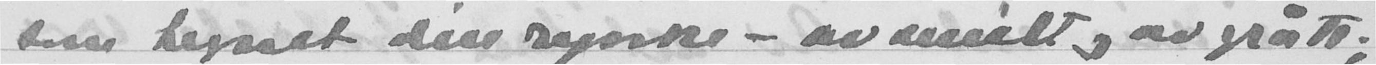som tegnet din rynke - av ansikt og av grått;
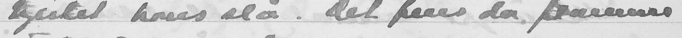hjertet hans slå. Det fins da flamme
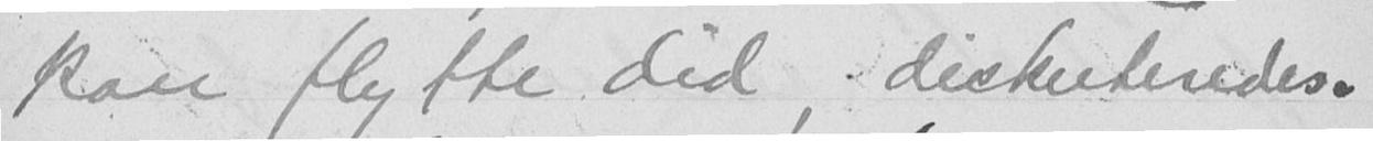kan flytte did, diskuteredes.
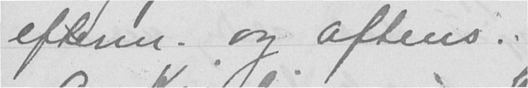efterm. og aftens.
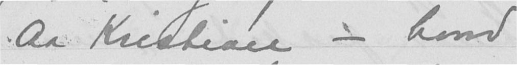Aa Kristian - hvad
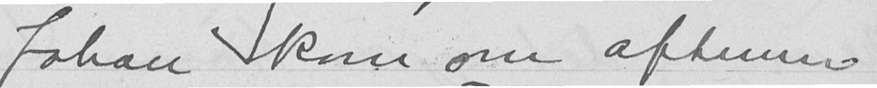Johan kom om aftenen
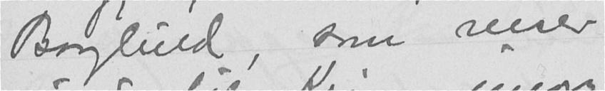Borghild som reiser
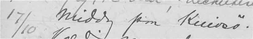17/10 Middag paa Knivsø.
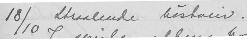18/10 Straalende høstveir.
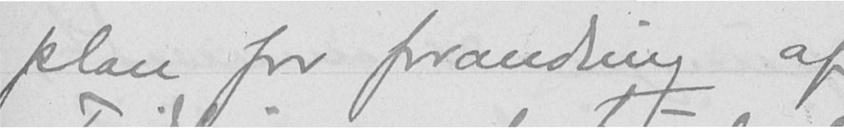plan for forandring af
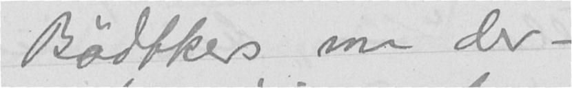Bødtkers var der -
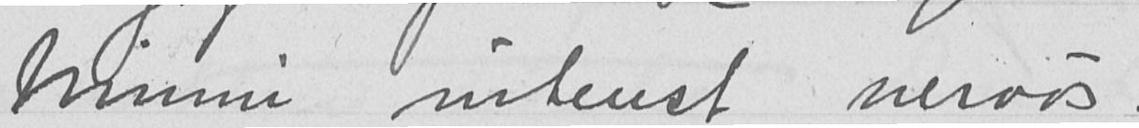Minni intenst nervøs.
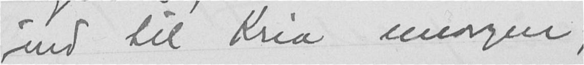ind til Kria imorgen,
